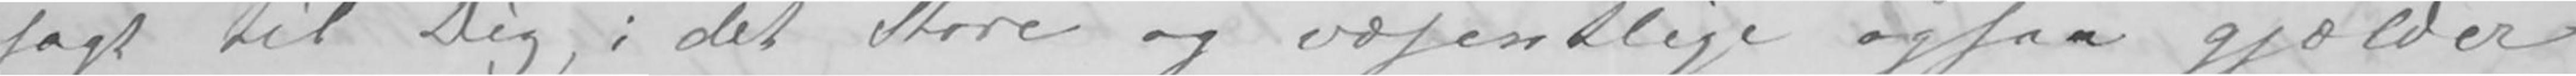sagt til Dig, i det Store og væsentlige ogsaa gjælder
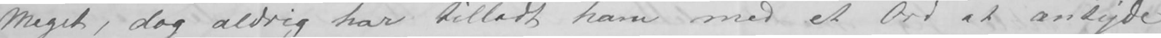Meget, dog aldrig har tilladt ham med et Ord at antyde
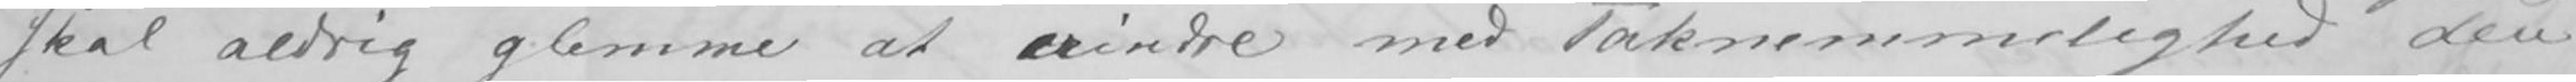skal aldrig glemme at erindre med Taknemmelighed den
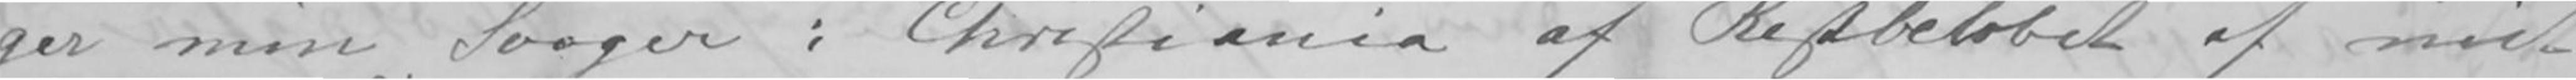ger min Svoger i Christiania af Restbeløbet af mit
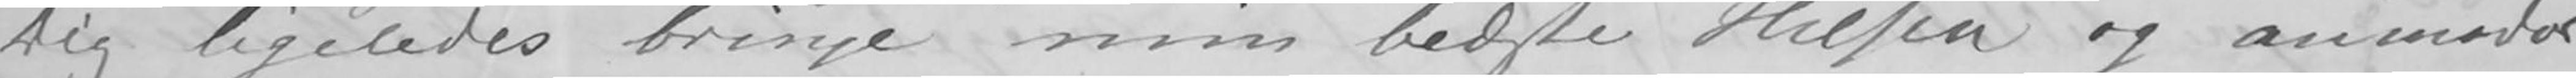Dig ligeledes bringe min bedste Hilsen og anmoder
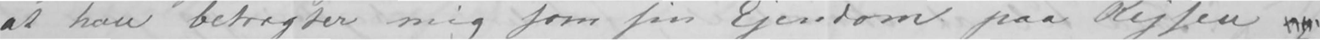at han betragter mig som sin Ejendom paa Rejsen og
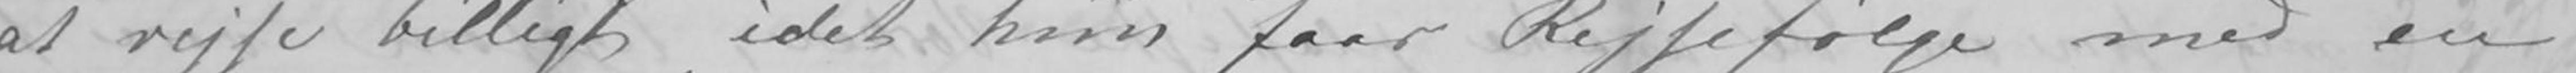at rejse billigt idet hun faar Rejsefølge med en
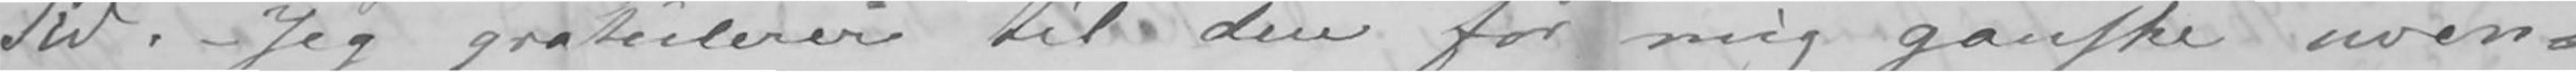Tid.- Jeg gratulerer til den for mig ganske uven-
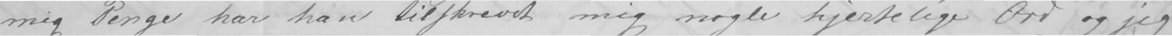mig Penge har han tilskrevet mig nogle hjertelige Ord og jeg
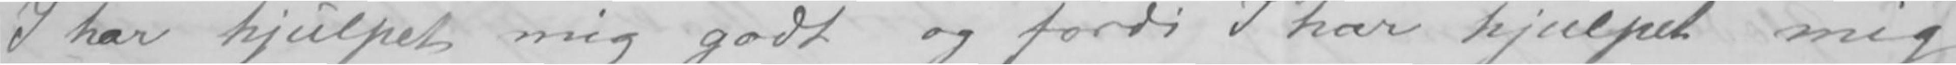I har hjulpet mig godt og fordi I har hjulpet mig
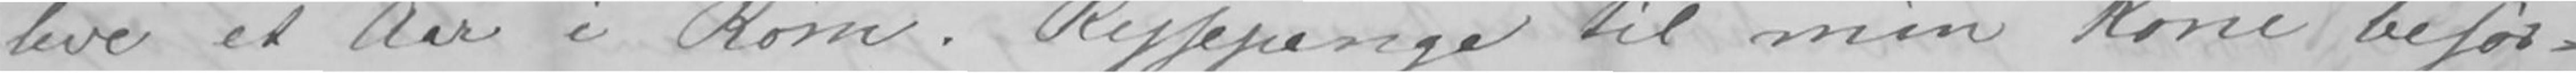leve et Aar i Rom. Rejsepenge til min Kone besør-
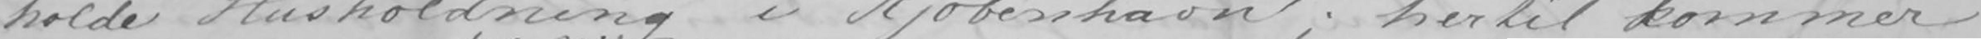holde Husholdning i Kjøbenhavn; hertil kommer
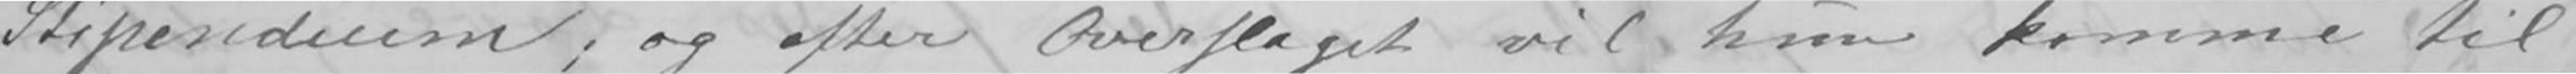Stipendium; og efter Overslaget vil hun komme til
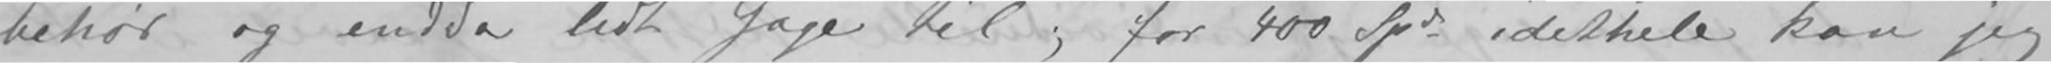behør og endda lidt Gage til; for 400 Spd idethele kan jeg
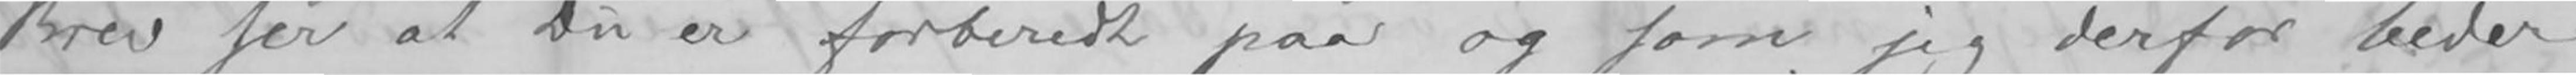Brev ser at Du er forberedt paa og som jeg derfor beder
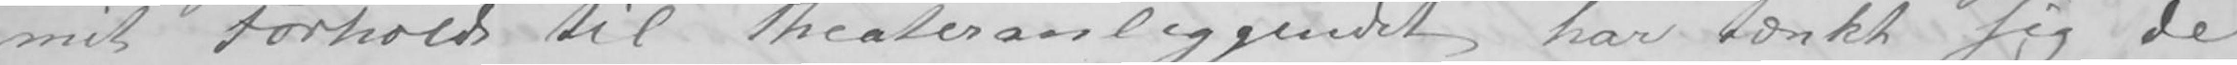mit Forhold til Theateranliggendet har tænkt sig de
